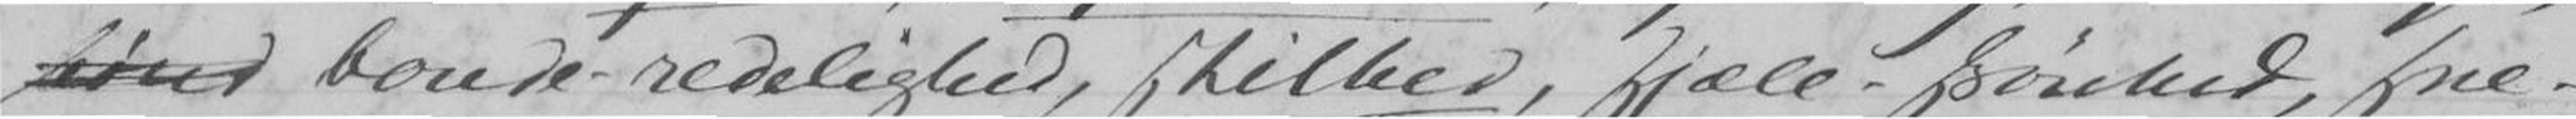bonde-redelighed, stilhed, sjæle-skønhed, sne-
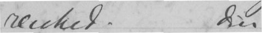renhed.Din
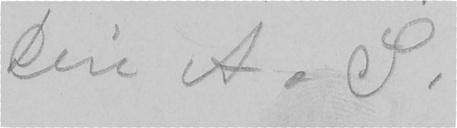Din A.S.
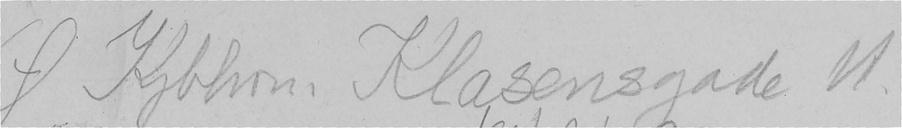Ø Kjbhvn. Klasensgade 11.
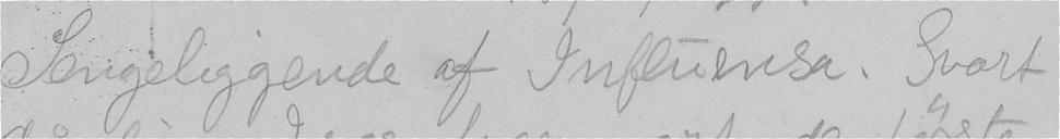Sengeliggende af Influensa. Svært
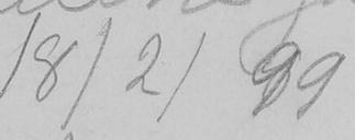18/2/99
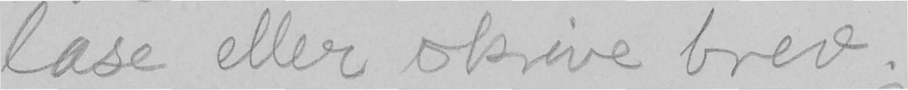læse eller skrive brev.
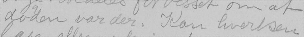døden var der. Kan hverken
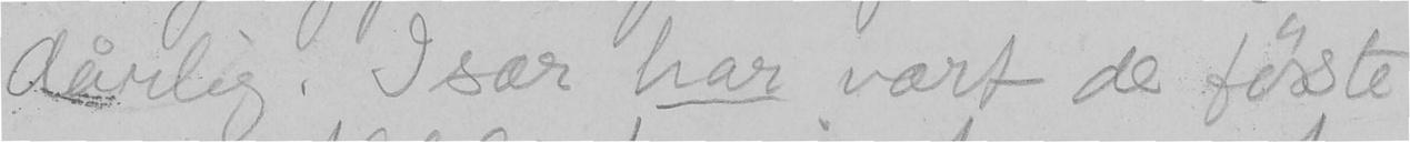dårlig. Især har vært de første
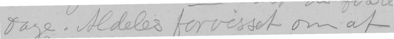dage. Aldeles forvisset om at
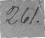261.
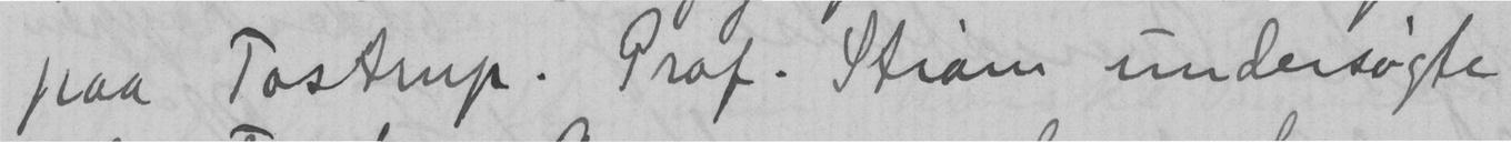paa Tostrup. Prof. Strøm undersøgte
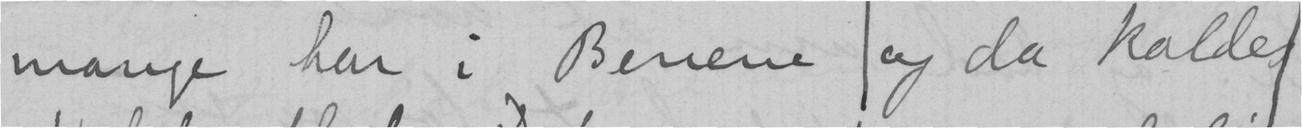mange har i Benene og da kalder
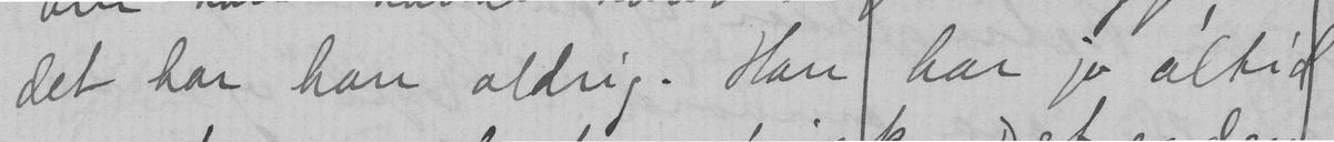det har han aldrig. Han har jo altid
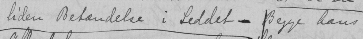liden Betændelse i Leddet - Begge hans
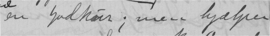en jodkur; men hjælper
